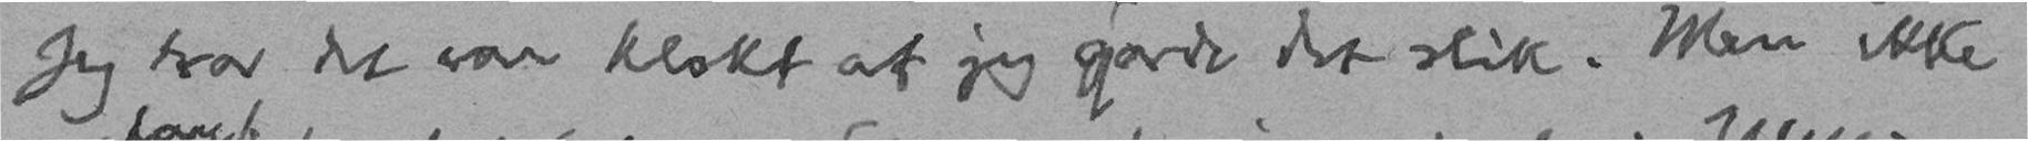Jeg tror det var klokt at jeg gjorde det slik. Men ikke
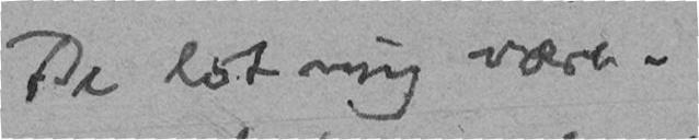De lot mig være.
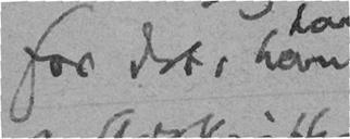for det, han
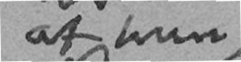at hun
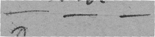- - -
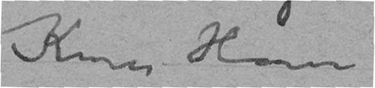Knut Hamsun
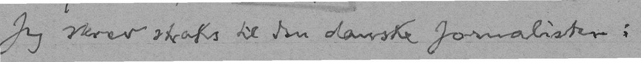Jeg skrev straks til den danske Jornalisten:
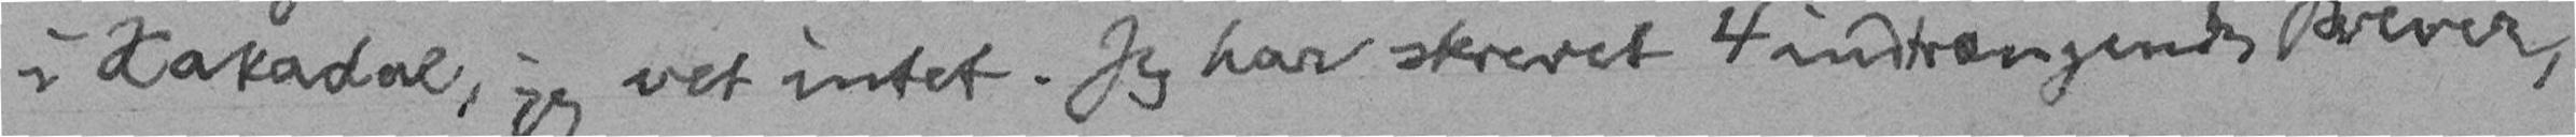i Hakadal, jeg vet intet. Jeg har skrevet 4 indtrængende Brever,
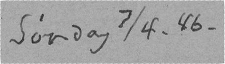Søndag 7/4. 46.
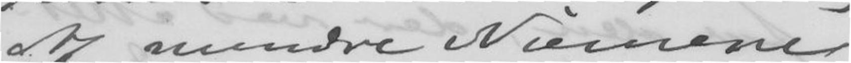Af mindre Numere
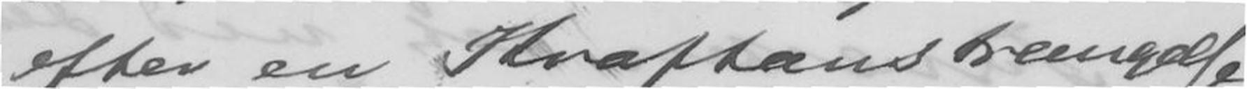efter en Kraftanstrængelse,
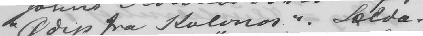"Ødip fra Kolonos". Solda-
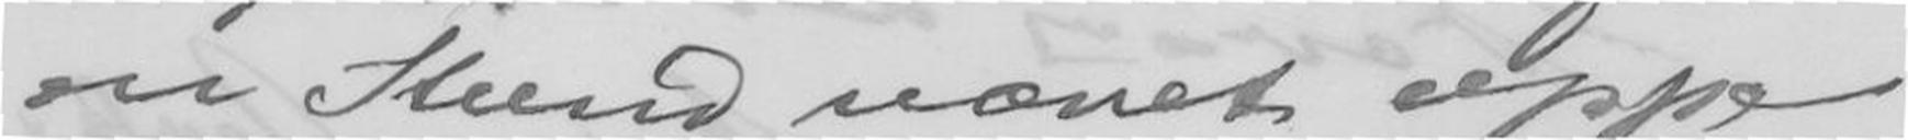en Stund været oppa
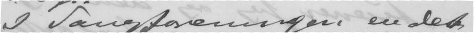I Sangforeningen er det
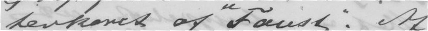terkoret af "Faust". Af
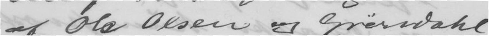af Ole Olsen og Grøndahl
